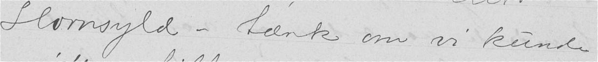Hornsyld – tænk om vi kunde
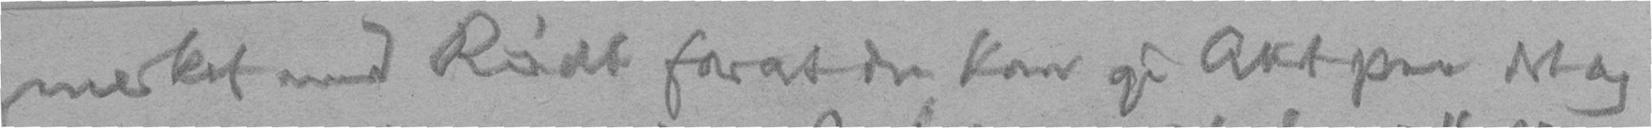merket med Rødt forat du kan gi Akt paa det og
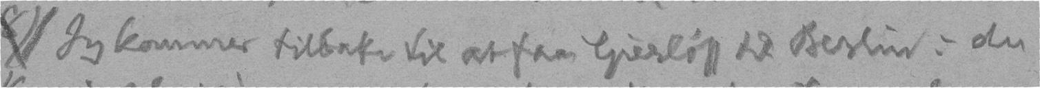8) Jeg kommer tilbake til at faa Gierløff til Berlin: du
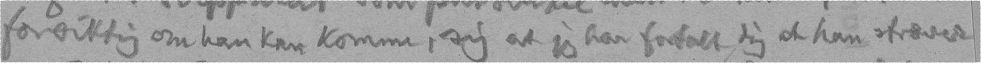forsiktig om han kan komme, sig at jeg har fortalt dig at han stræver
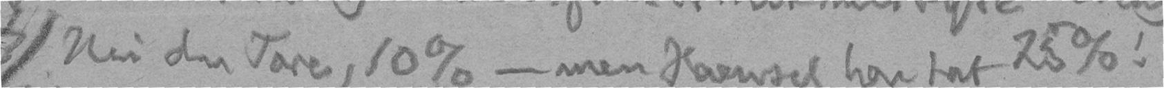7) Nei du Tore, 10% - men Haensel har tat 25%!
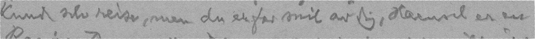kunde selv reise, men du er for snil av dig, Haensel er en
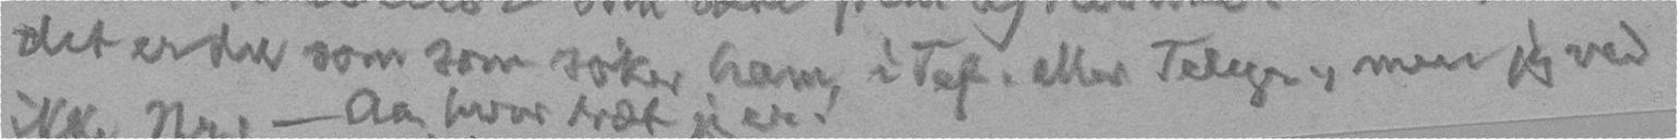det er du som som søker ham, i Tef. eller Telegr., men jeg ved
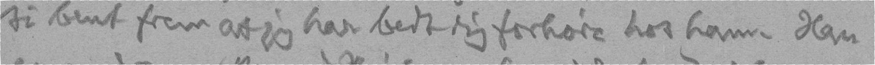si bent frem at jeg har bedt dig forhøre hos ham. Han
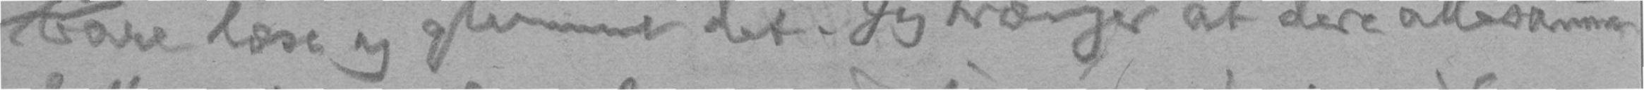bare læse og glemme det. Jeg trænger at dere allesammen
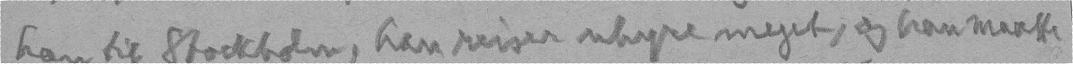han til Stockholm, han reiser uhyre meget, og han maatte
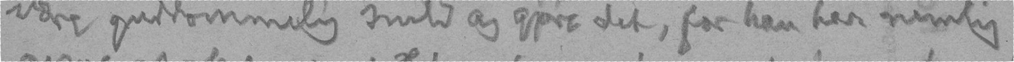være guddommelig snild og gjøre det, for han har nemlig
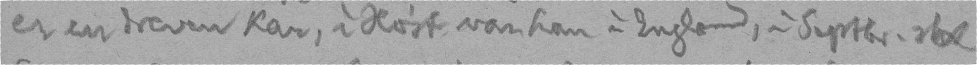er en dreven Kar, i Høst var han i England, i Septbr. skal
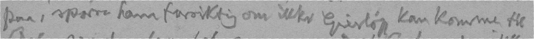paa, spørre ham forsiktig om ikke Gierløff kan komme til
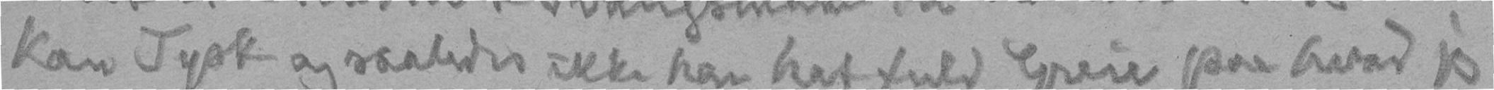kan Tysk og saaledes ikke har hat fuld Greie paa hvad jeg
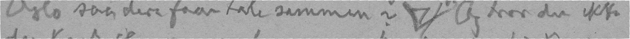Oslo saa dere faar tale sammen? 7) Og tror du ikke
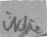ikke
